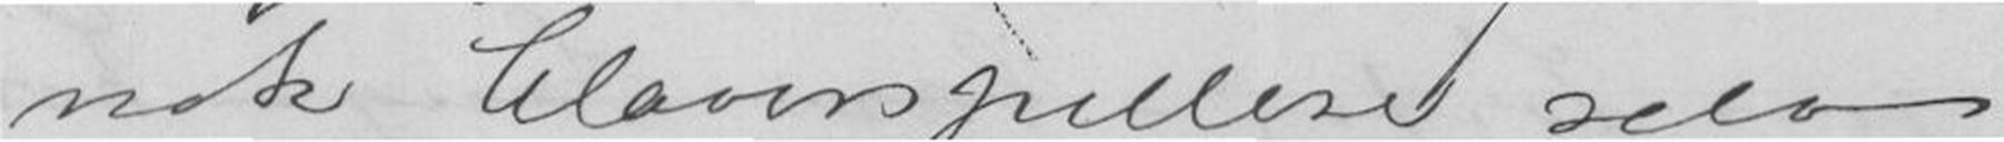nok Claverspillere selv
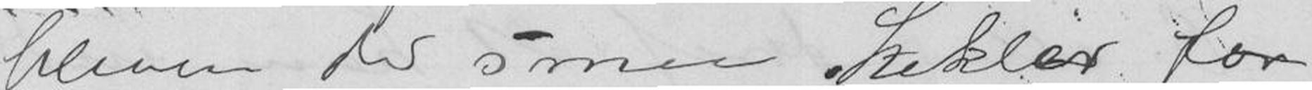bleven de smaa Stekler for
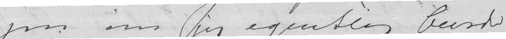paa om jeg egentlig burde
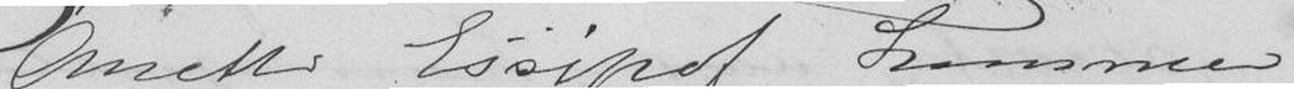Anette Essipof kommer
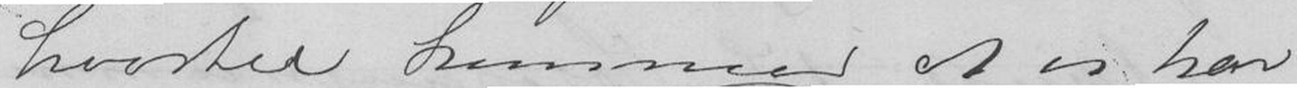hvortil kommer at vi har
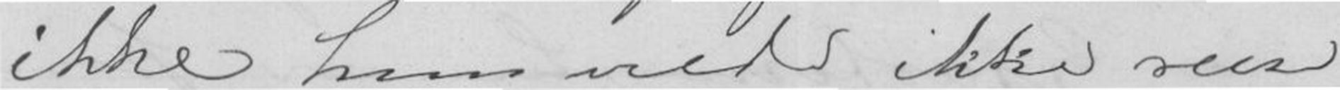ikke hun vilde ikke reise
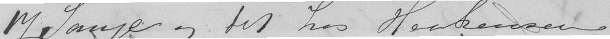17 Sange og det hos Haakonsen.
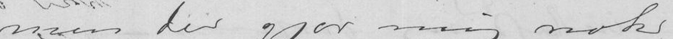men Du gjør mig nok
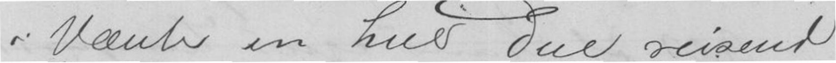i Vænte en heel Deel reisend
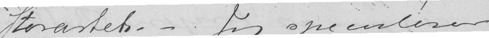Storartet. - Jeg speculerer
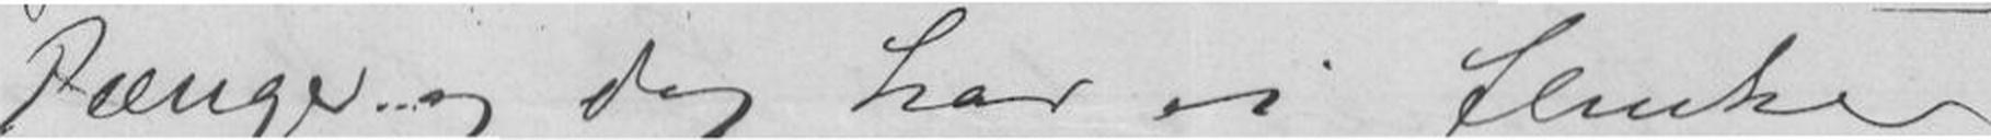Pænge og dog har vi flinke
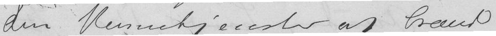den Vennetjenste at brænde
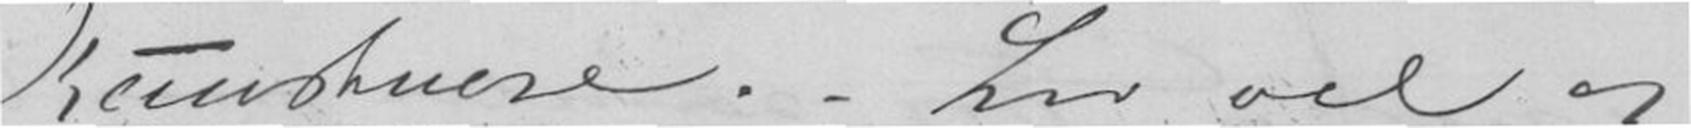Kunstnere. - Lev vel og
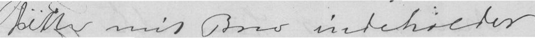dette mit Brev indeholder
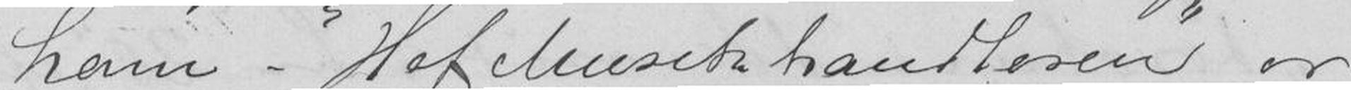ham - "Hof Musikhandleren" er
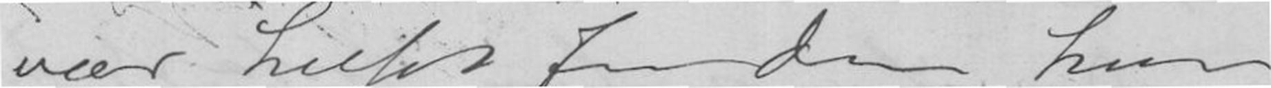vær hilset fra Din heng
